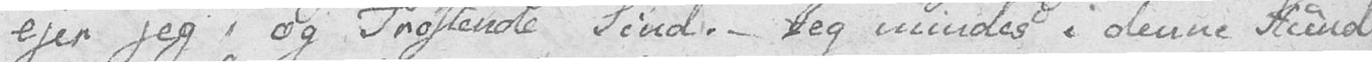ejer jeg, og Trøstende Sind. – Jeg mindes i denne Stund
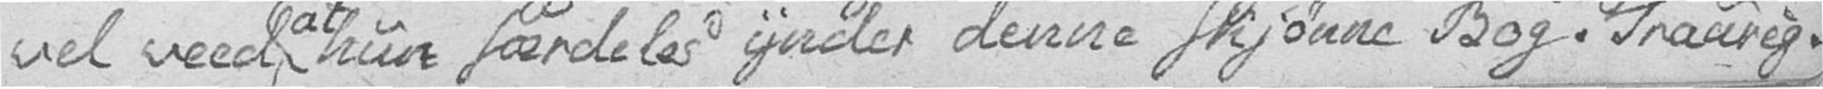vel veed at hun særdeles ynder denne skjønne Bog. Traurig.
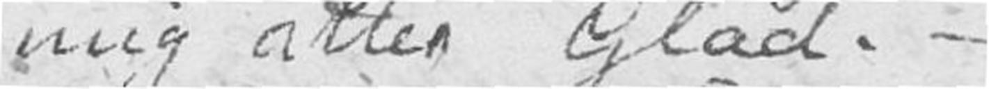mig atter Glad. –
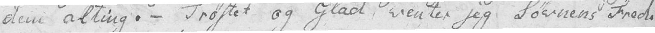dem alting. – Trøstet og Glad, venter jeg Søvnens Fred.
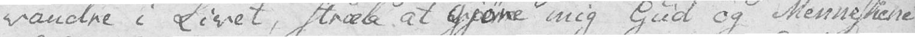vandre i Livet, stræbe at gjøre mig Gud og Menneskene
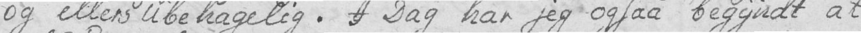og ellers ubehagelig. I Dag ar jeg ogsaa begyndt at
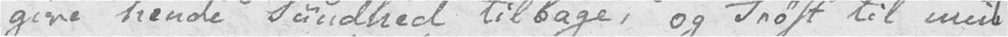give hende Sundhed tilbage, og Trøst til mit
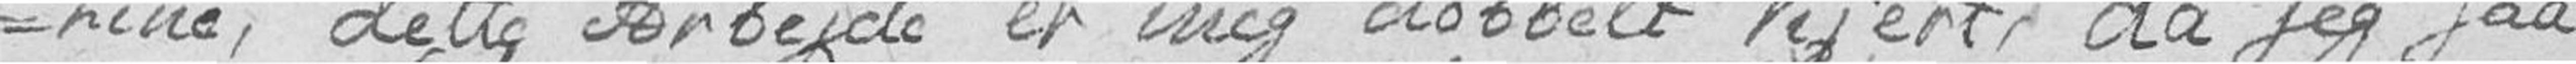-rine, dette Arbejde er mig dobbelt kjert, da jeg saa
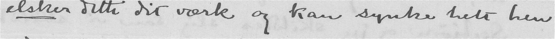elsker dette dit værk og kan synke helt hen
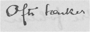Ofte tænker
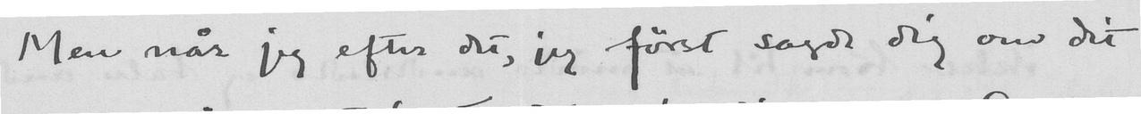Men når jeg efter det, jeg først sagde dig om det
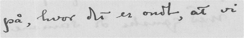på, hvor det er ondt, at vi
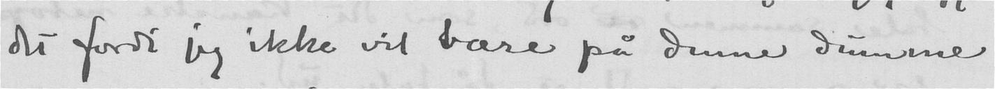det fordi jeg ikke vil bære på denne dumme
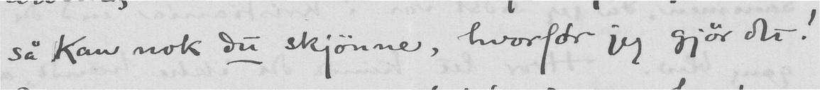så kan nok du skjønne, hvorfor jeg gjør det!
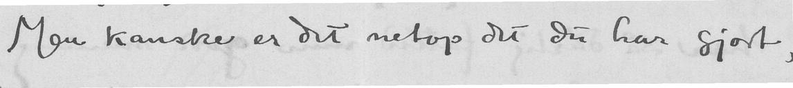Men kanske er det netop det du har gjort,
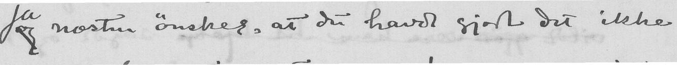og næsten ønsker, at du havde gjort det ikke
